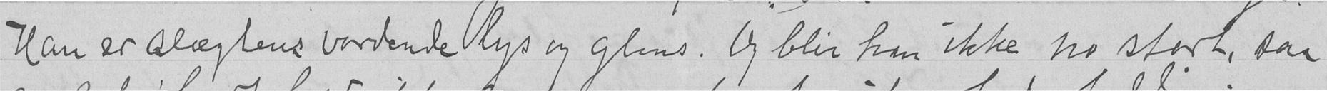Han er slægtens vordende lys og glans. Og blir han ikke no stort, saa
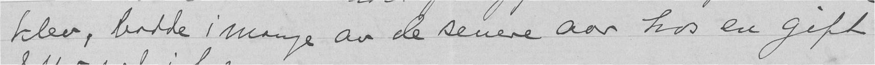klev, bodde i mange av de senere aar hos en gift
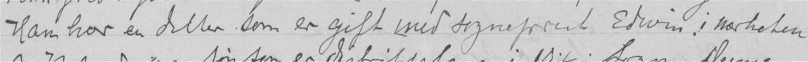Han har en datter som er gift med sogneprest Edwin i nærheten
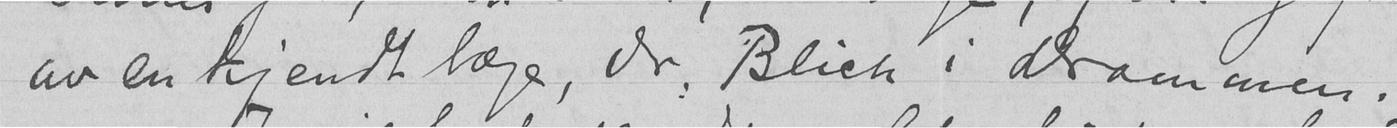av en kjendt læge, dr. Blich i Drammen.
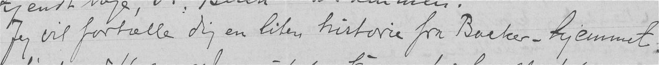Jeg vil fortælle dig en liten historie fra Backer-hjemmet.
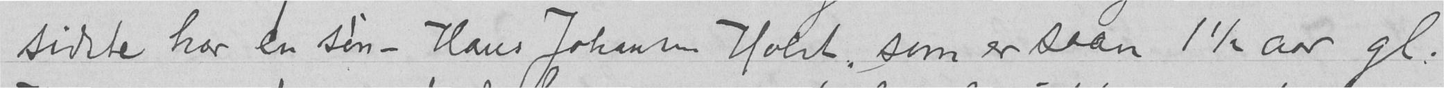sidste har en søn - Hans Johansen Holst, som er saan 1 ½ aar gl.
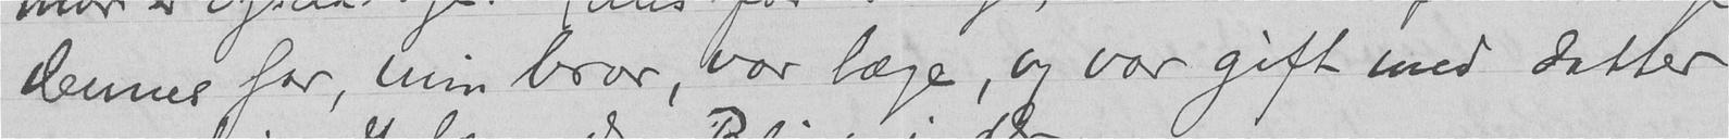dennes far, min bror, var læge, og var gift med datter
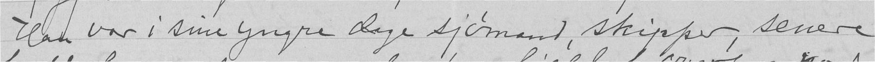Han var i sine yngre dage sjømand, skipper, senere
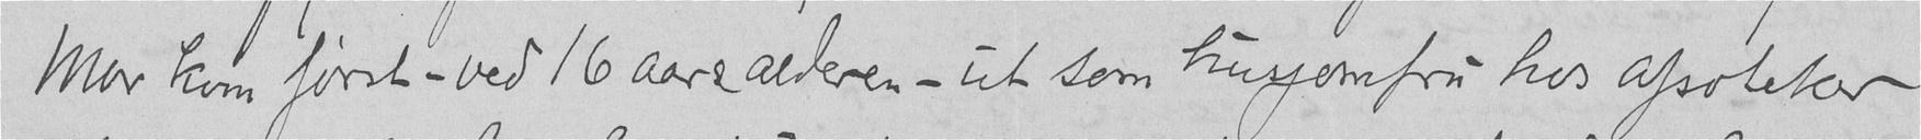Mor kom først - ved 16 aars alderen - ut som husjomfru hos apoteker
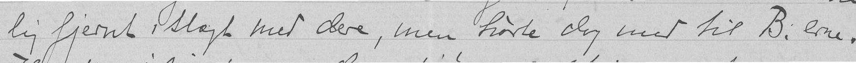lig fjernt i slægt med dere, men hørte dog med til B.erne.
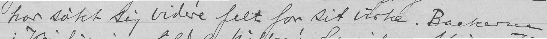har søkt sig videre felt for sit virke. Backerne
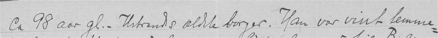Ca 98 aar gl. - Hstrands ældste borger. Han var visst temme-
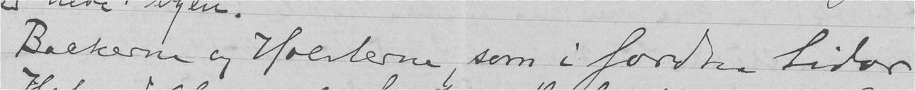Backerne og Holsterne, som i fordne tider
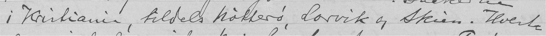i Kristiania, tildels Nøtterø, Larvik og Skien. Holst-
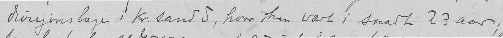divisjonslæge i Kr.sand S, hvor han vært i snart 23 aar;
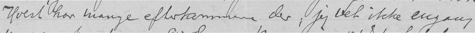Holst har mange efterkommere der; jeg vet ikke engang
